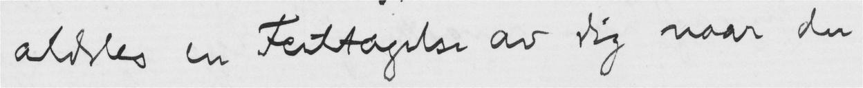aldeles en Feiltagelse av dig naar du
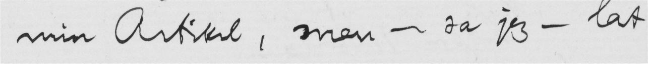min Artikel, men - sa jeg - lat
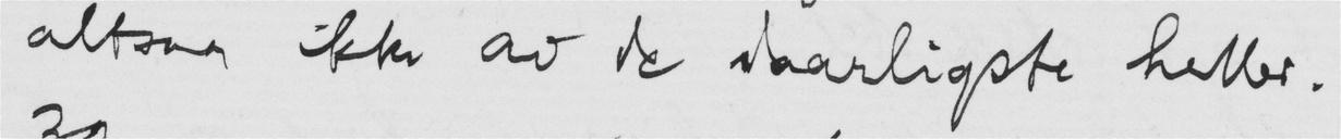altsaa ikke av de daarligste heller.
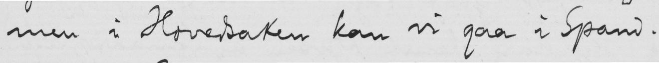men i Hovedsaken kan vi gaa i Spand.
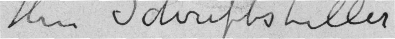Hrn Schriftsteller
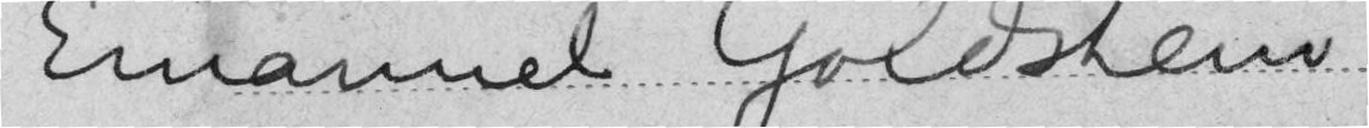Emanuel Goldstein
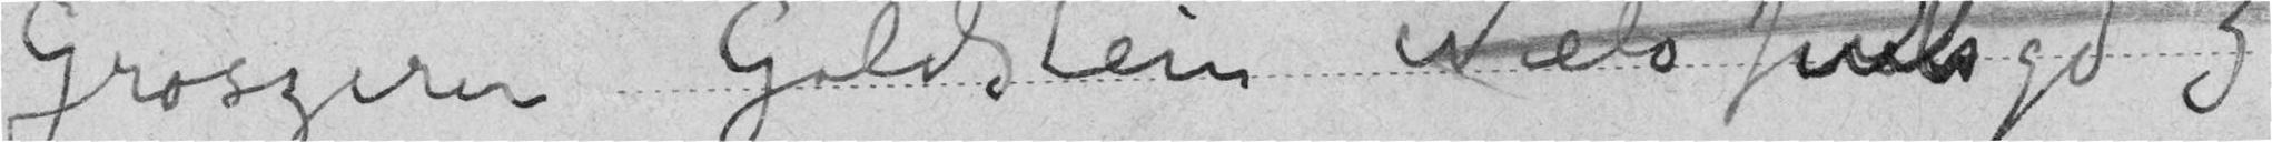Groszerer Goldstein Niels Juels gd 3
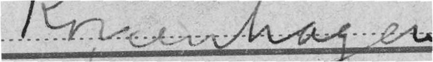Kopenhagen
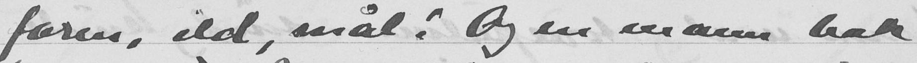form, ild, mål; Og en mann bak
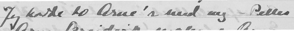Jeg hadde to Arne'r med mig - Pelles
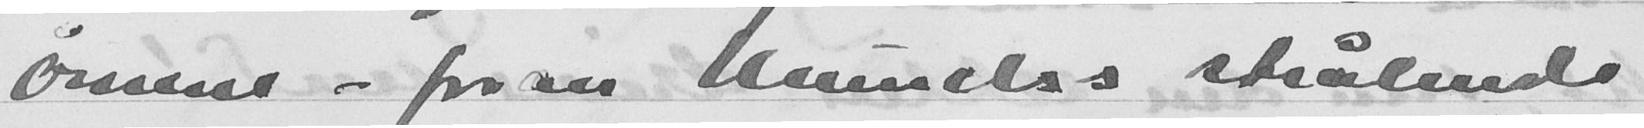øinene - foren Munchs strålende
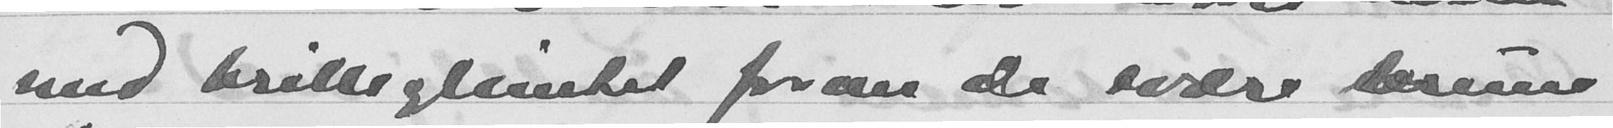med brilleglimtet foran de svære brune
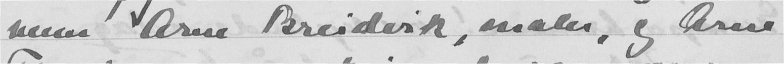venn Arne Breidvik, maler, og Arne
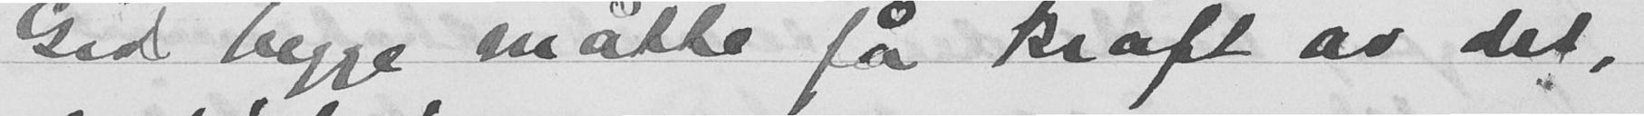Gid begge måtte få kraft av det,
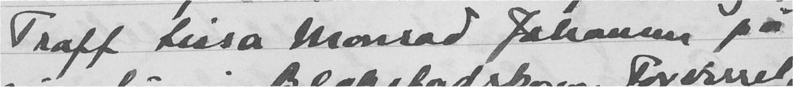Traff Lisa Monrad Johansen på
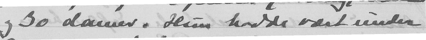og 30 damer. Hun hadde vært under
